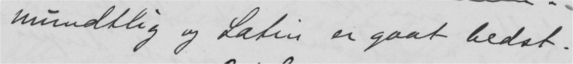mundtlig og Latin er gaat bedst.
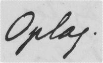Oplag.
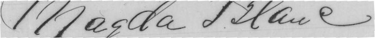Magda Blanc
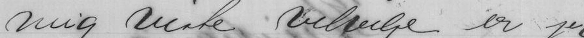mig viste velvilje er jeg
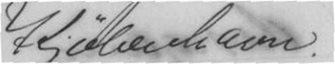Kjøbenhavn
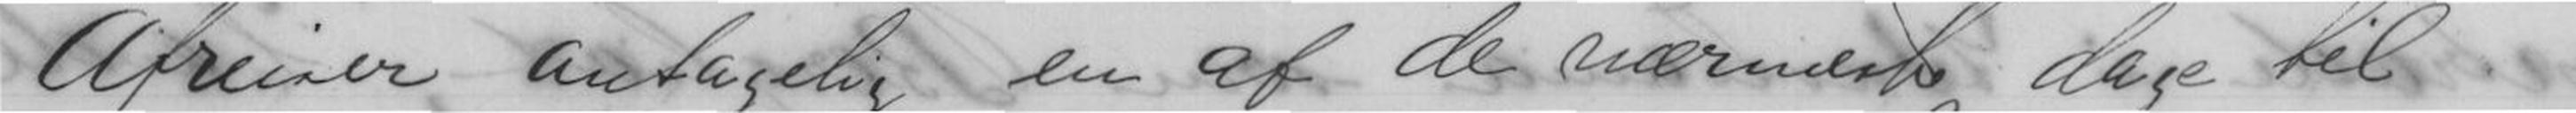Afreiser antagelig en af de nærmeste dage til
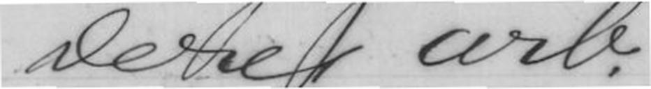Deres ærb.
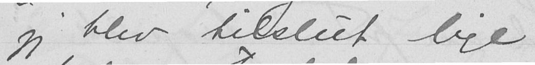jeg blev tilslut lige
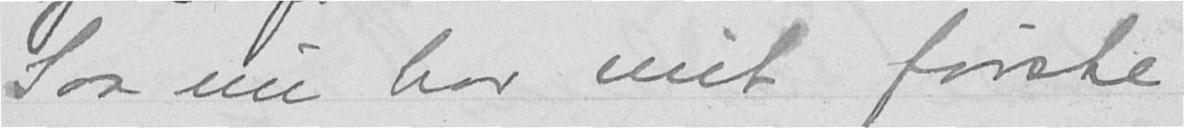Saa nu har mit første
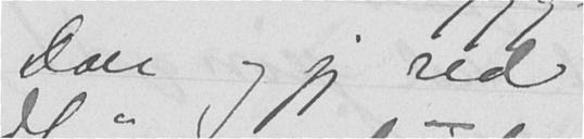Don og jeg red
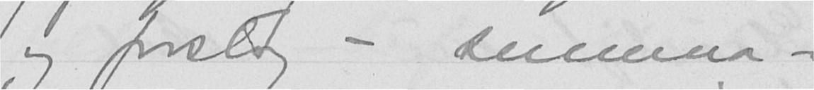og forslag - summa-
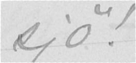sjø!
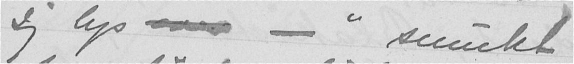sig lys over -" smukt
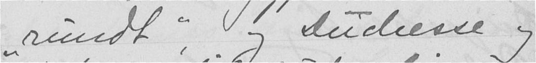"rundt", og Duchesse og
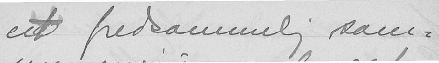et fredsommelig sam-
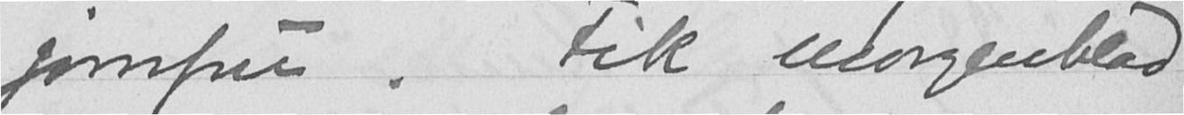jomfru. Fik morgenblad
